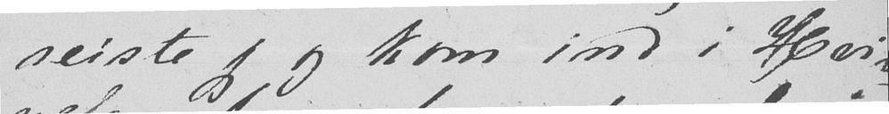reiste jeg og kom ind i Hvir-
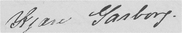Kjære Garborg.
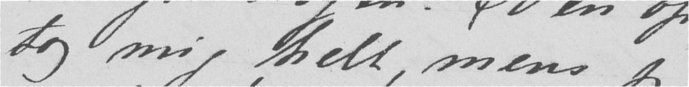tog mig helt, mens jeg
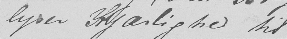lyser Kjærlighed til
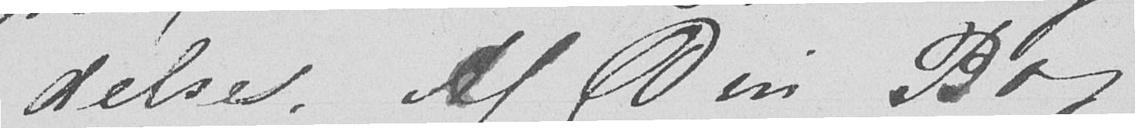delse. Af Din Bog
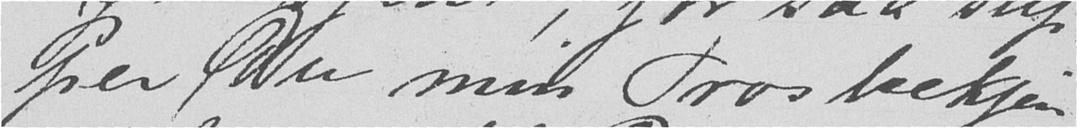per Du min Trosbekjen
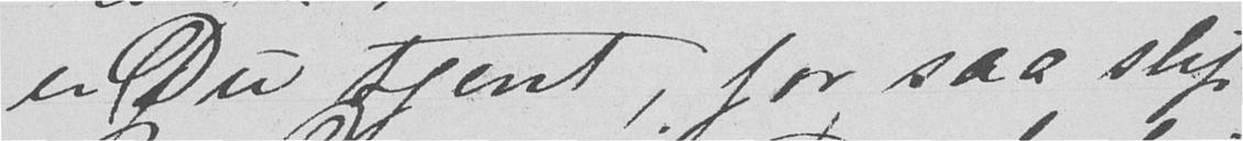er Du tjent, for saa slip-
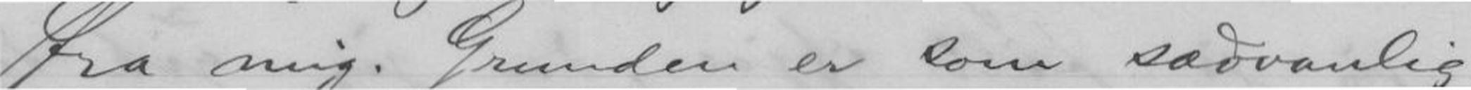fra mig. Grunden er som sædvanlig
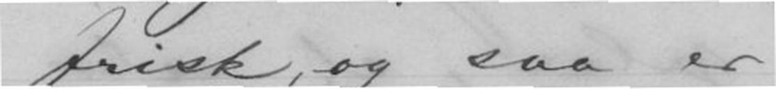frisk, og saa er
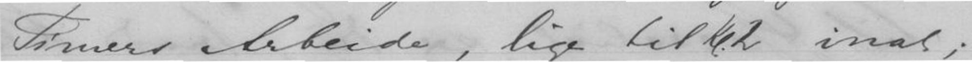Timers Arbeide, lige til kl.2 inat;
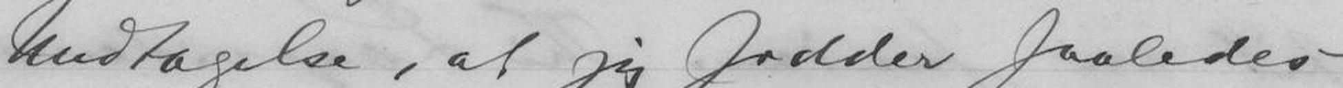Undtagelse, at jeg sidder saaledes
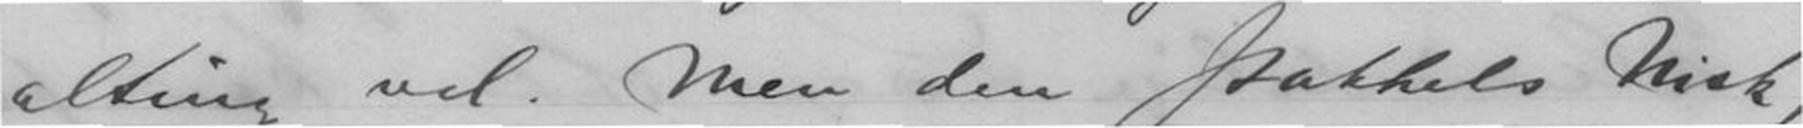alting vel. Men den stakkels Nick,
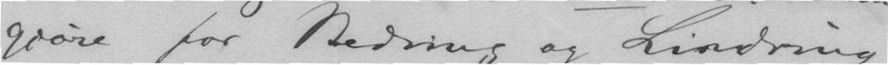gjøre for Bedring og Lindring!
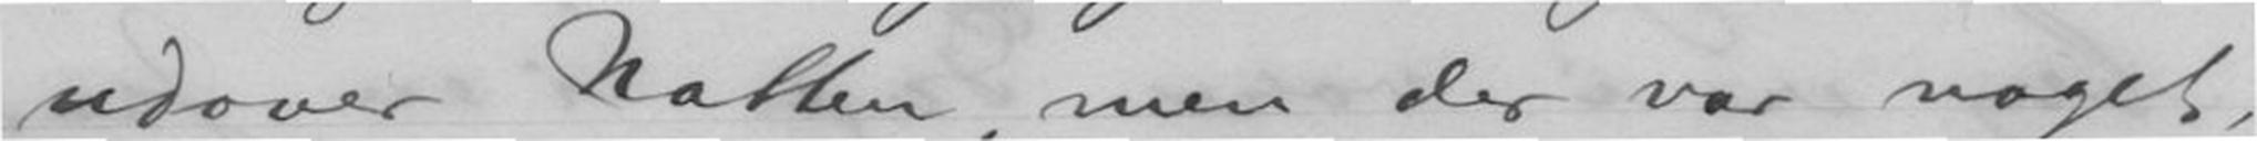udover Natten, men der var noget,
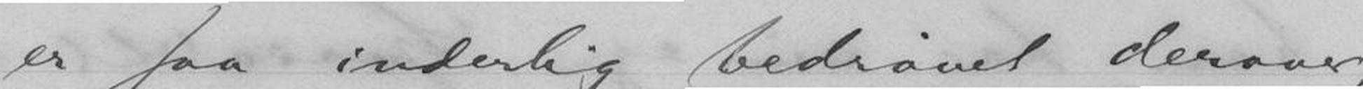er saa inderlig bedrøvet derover,
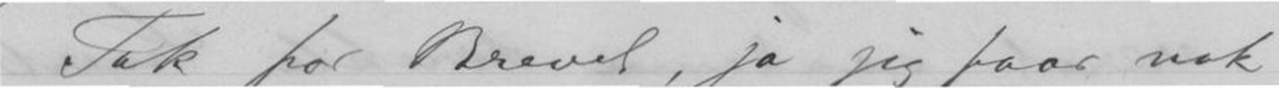Tak for Brevet, ja jeg faar nok
